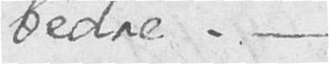bedre. –
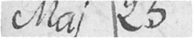Maj 25
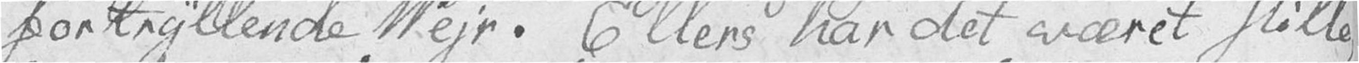fortryllende Vejr. Ellers har det været stille
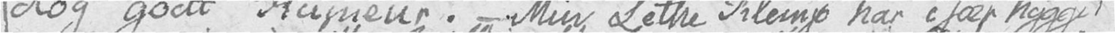dog godt Humeur. – Min Lethe Klemp har især hygget
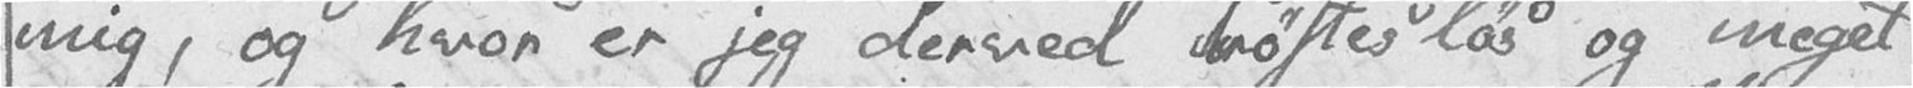mig, og hvor er jeg derved trøstesløs og meget
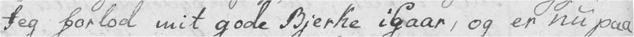Jeg forlod mit gode Bjerke iGaar, og er nu paa
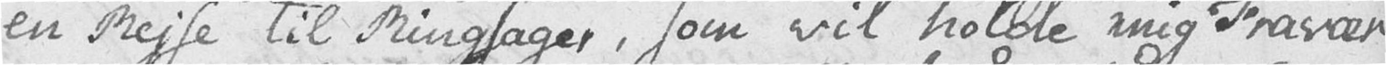en Rejse til Ringsager, som vil holde mig Fravær
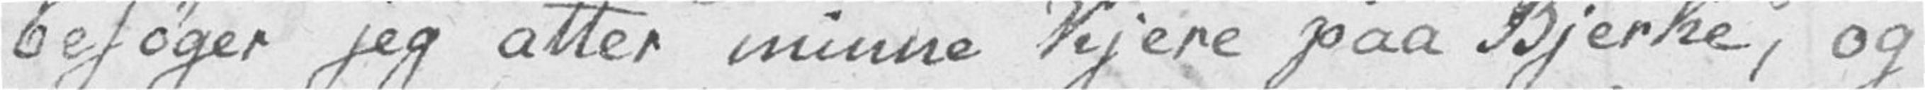besøger jeg atter minne Kjere paa Bjerke, og
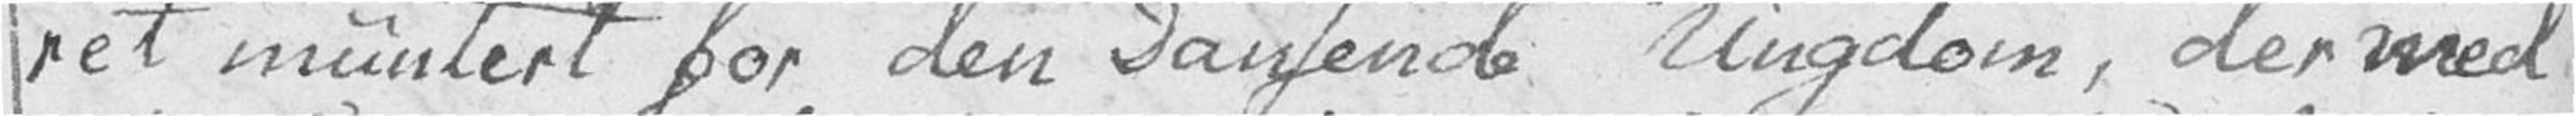ret muntert for den Dansende Ungdom, der med
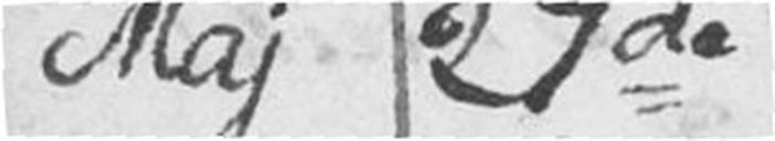Maj 27de
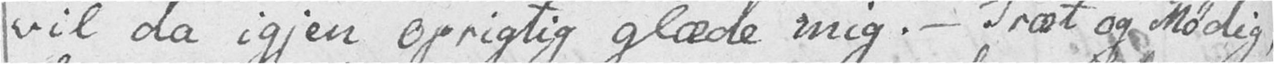vil da igjen oprigtig glæde mig. – Træt og Mødig,
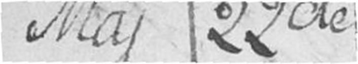Maj 22de
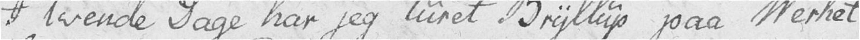I tvende Dage har jeg turet Bryllup paa Verket
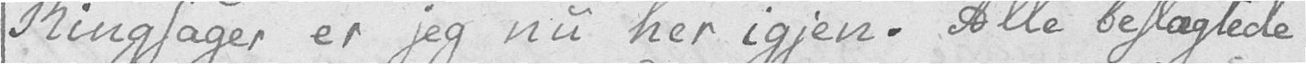Ringsager er jeg nu her igjen. Alle beslægtede
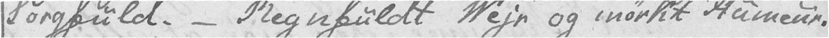Sorgfuld. – Regnfuldt Vejr og mørkt Humeur.
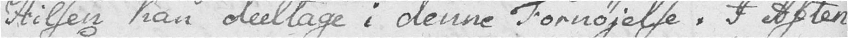Hilsen kan deeltage i denne Fornøjelse. I Aften
